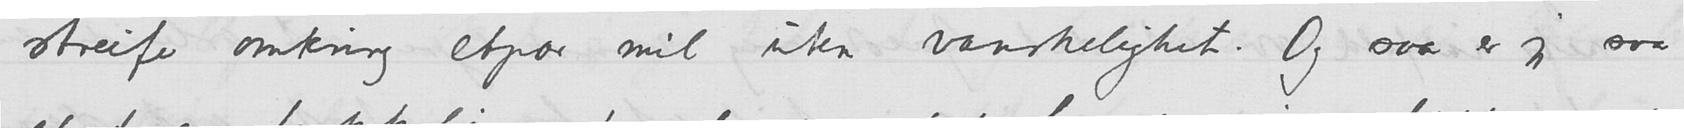at streife omkring etpar mil uten vanskelighet. Og saa er jeg saa
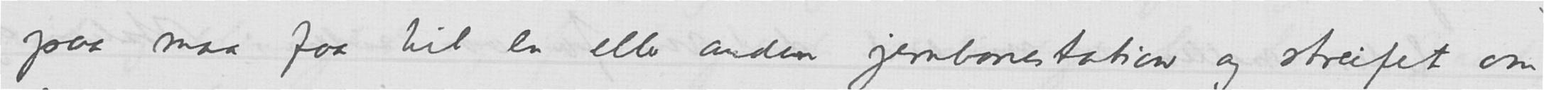paa maa faa til en eller anden jernbanestation og streifet om
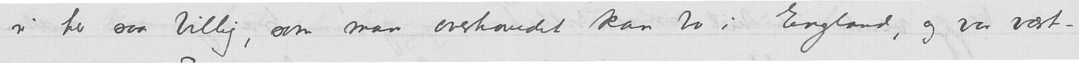vi her saa billig, som man overhovedet kan bo i England, og vor vært-
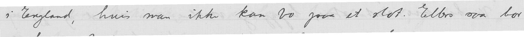i England, hvis man ikke kan bo paa et slot. Ellers saa bor
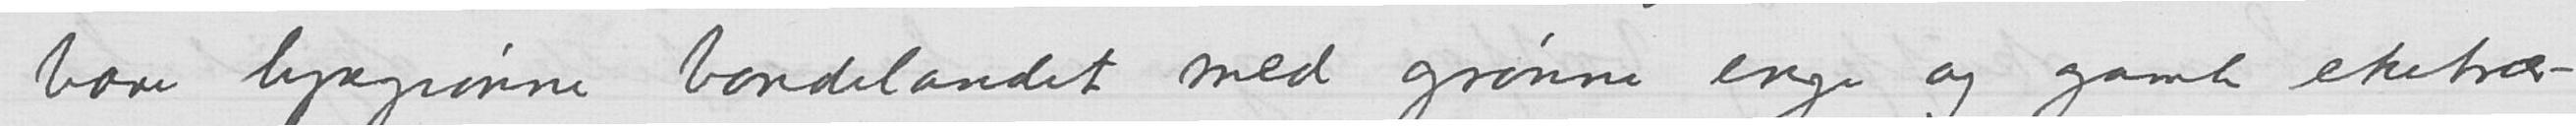bare lysegrønne bondelandet med grønne enger og gamle eketrær
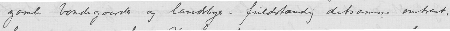gamle bondegaarde og landsbye - fuldstændig detsamme omtrent,
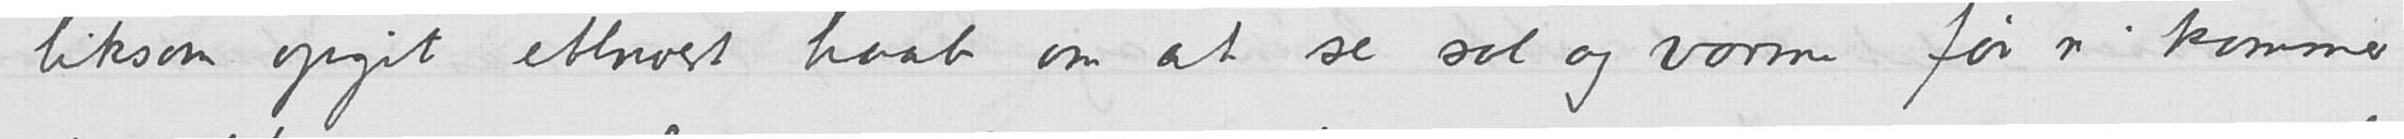liksom opgit ethvert haab om at se sol og varme før vi kommer
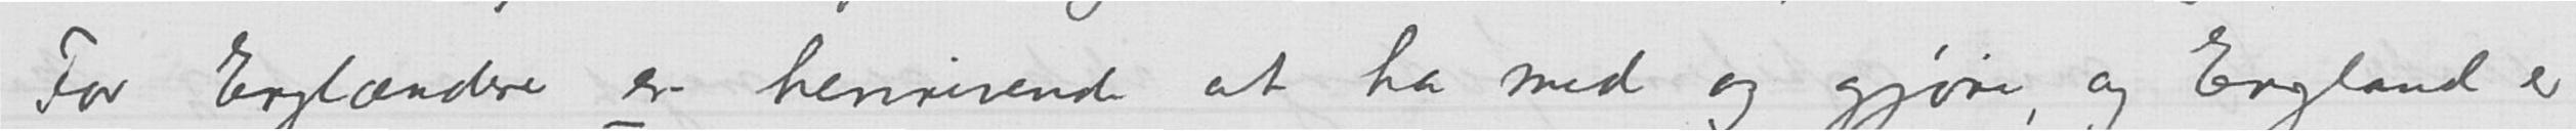For Englændere er henrivende at ha med og gjøre, og England er
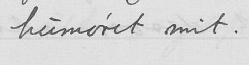humøret mit.
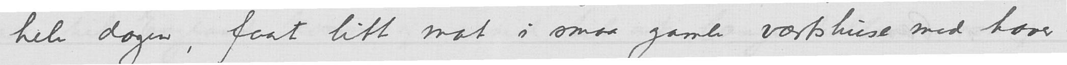hele dagen, faat lit mat i smaa gamle værtshuse med haver
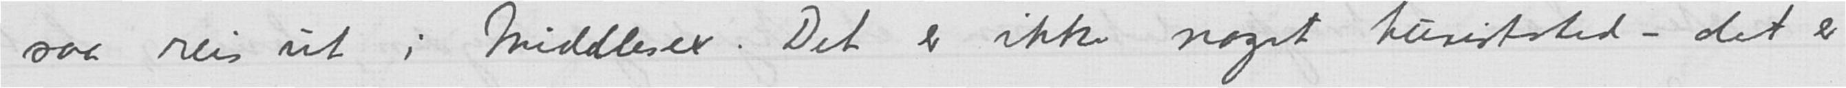saa reis ut i Middelsex. Det er ikke noget turiststed - det er
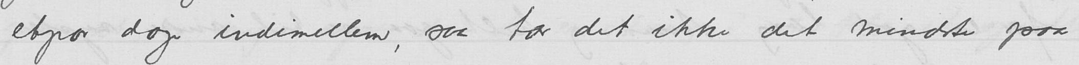etpar dage indimellem, saa tar det ikke det mindste paa
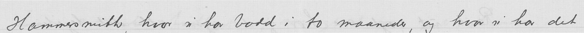Hammersmith, hvor vi har bodd i to maaneder, og hvor vi har det
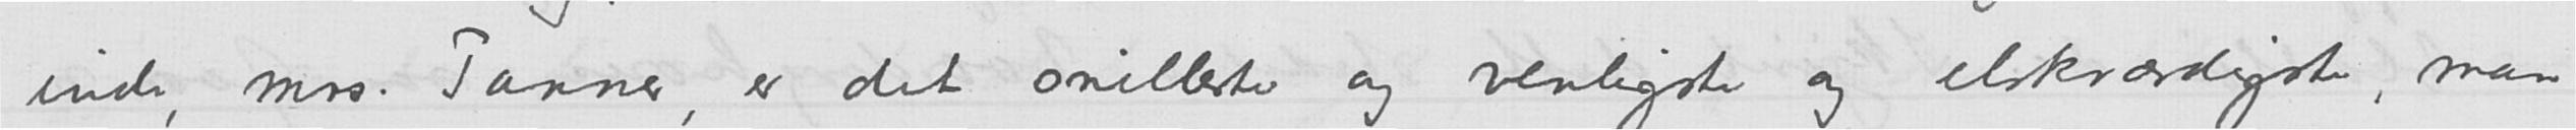inde, mrs. Tarner, er det snilleste og venligste og elskværdigste, man
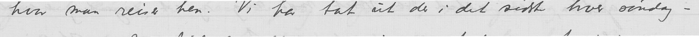hvor man reiser hen. Vi har tat ut der i det sidste hver søndag -
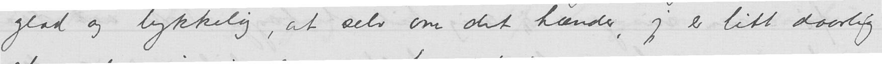glad og lykkelig, at selv om det hænder, jeg er litt daarlig
